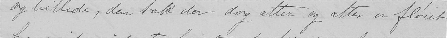og billede, den tak der dog atter og atter er fløiet
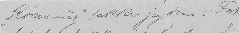"Rønnaug" takker jeg dem. For
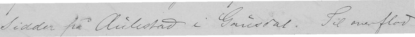sidder på Aulestad i Gausdal. Til overflod
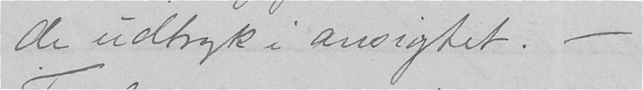de udtryk i ansigtet. -
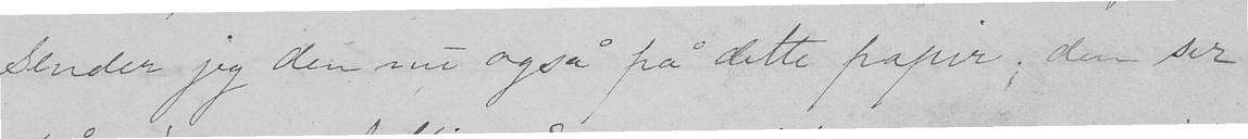sender jeg den nu også på dette papir; den ser
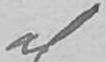al
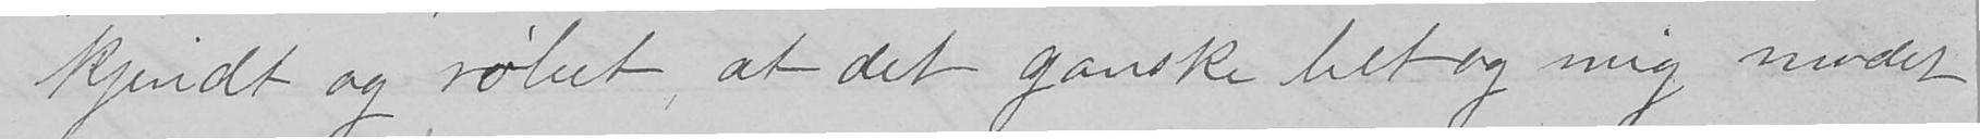kjendt og røbet, at det ganske betog mig modet
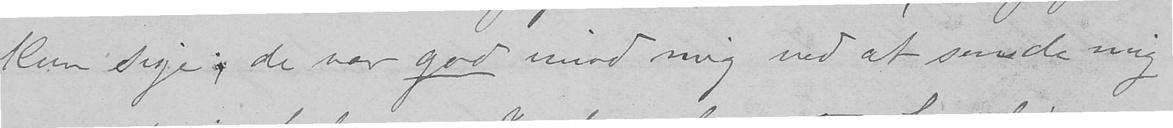kun sige; de var god imod mig ved at sende mig
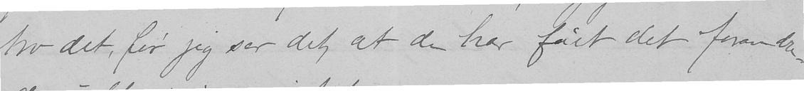tro det, før jeg ser det, at de har fået det forandre-
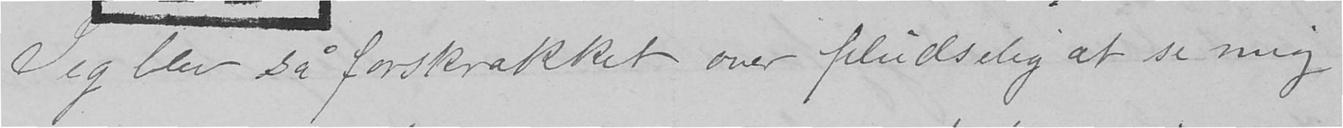Jeg blev så forskrækket over pludselig at se mig
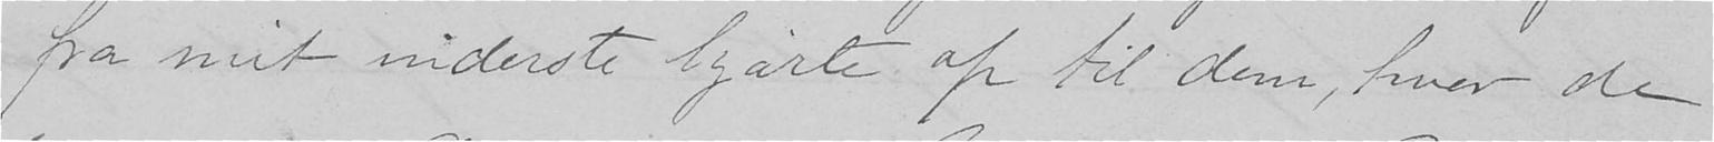fra mit inderste hjærte op til dem, hvor de
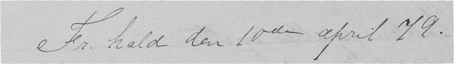Fr. hald den 10de april 79.
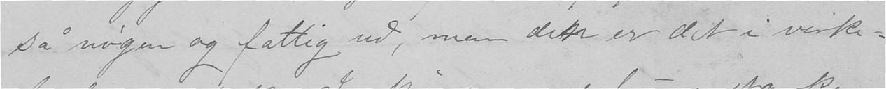så nøgen og fattig ud, men den er det i virke-
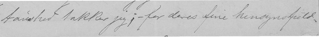taushed takker jeg; - for deres fine hensynsfuld-
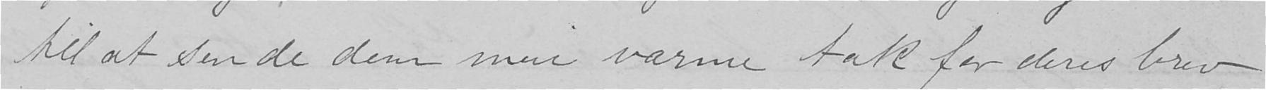til at sende dem min varme tak for deres brev
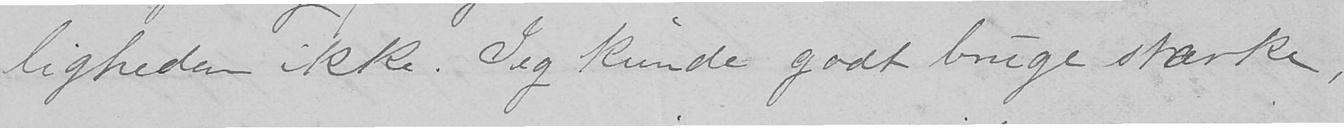ligheden ikke. Jeg kunde godt bruge stærke,
